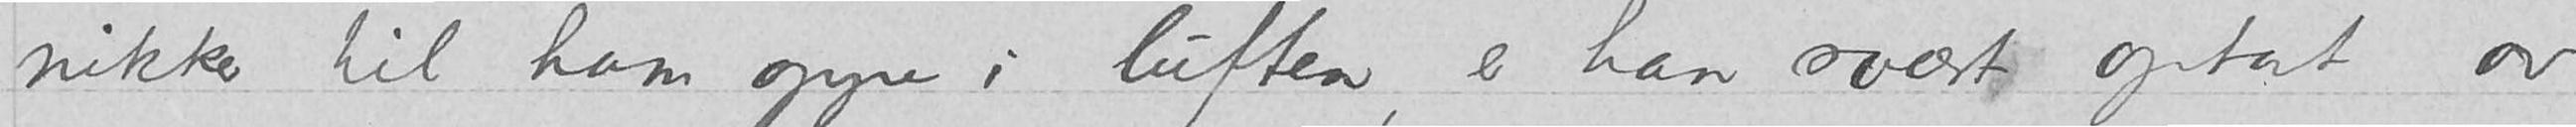nikker til ham oppe i luften, er han svært optat av
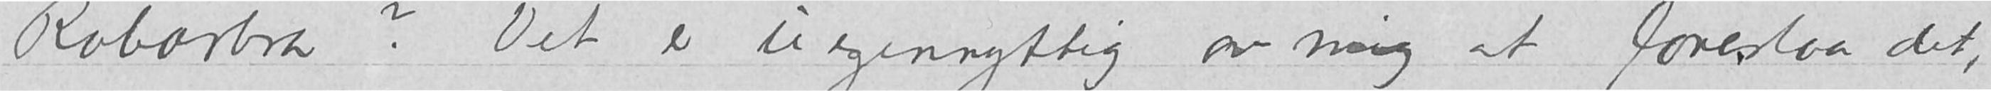Rabarbra? Det er uegennyttig av mig at foreslaa det,
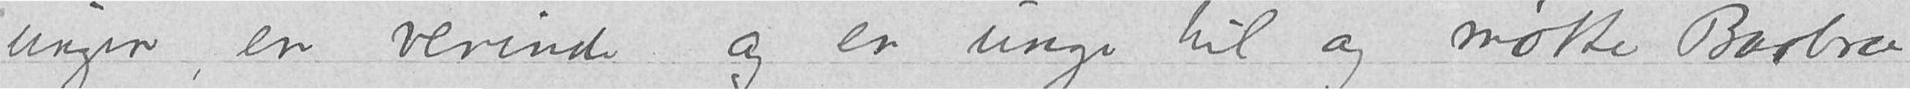ungen, en veninde og en unge til og møtte Barbra
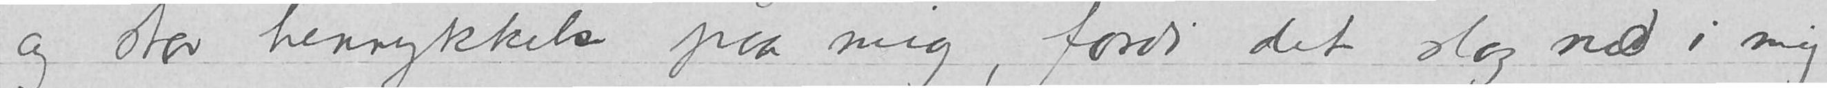og stor henrykkelse paa mig, fordi det slog ned i mig,
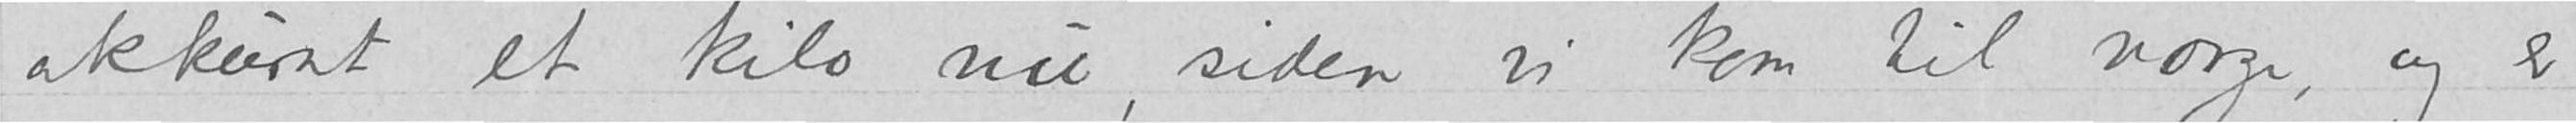akkurat et kilo nu, siden vi kom til norge , og er
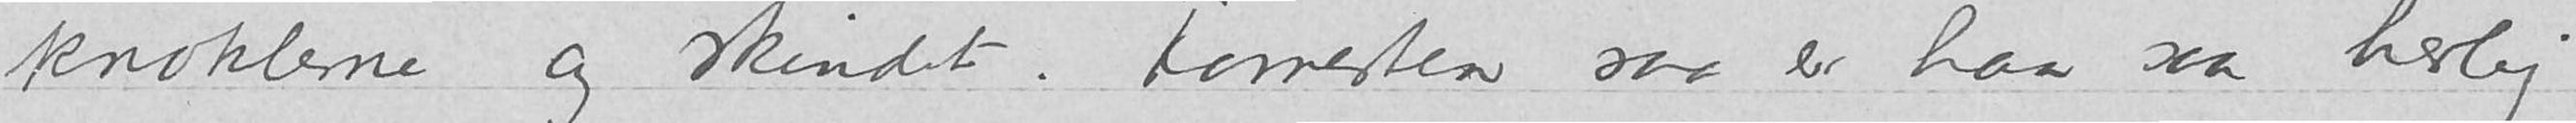knoklerne og skindet. Forresten saa er han saa herlig
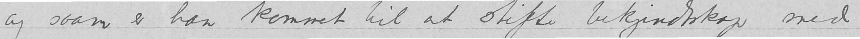og saan er han kommet til at stifte bekjendskap med
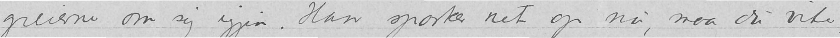greierne om sig igjen. Han sparker net op nu, maa du vite,
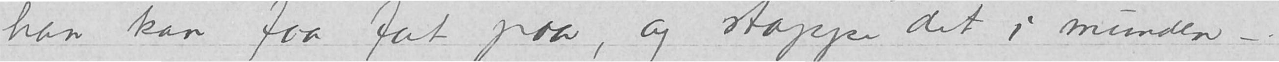han kan faa fat paa, og stapper det i munden –.
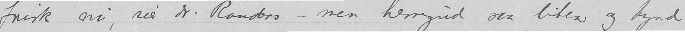frisk nu, sier dr. Randers – men herregud saa liten og tynd
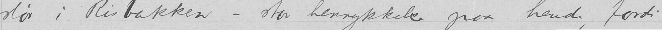slør i Risbakken – stor henrykkelse paa hende, fordi
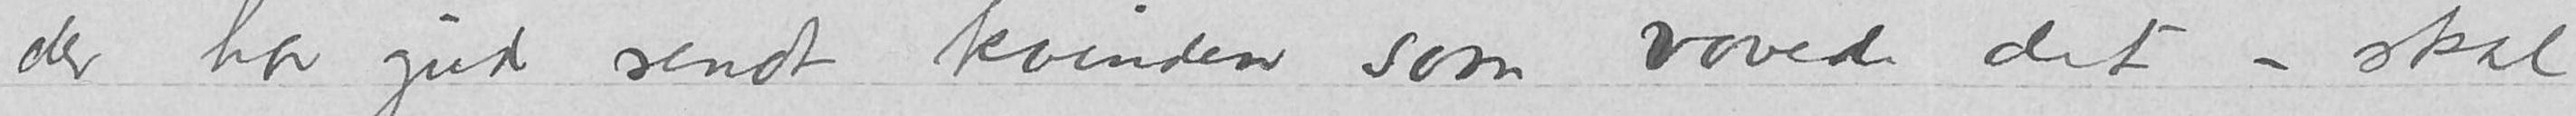der har gud sendt kvinden som vovede det – skal
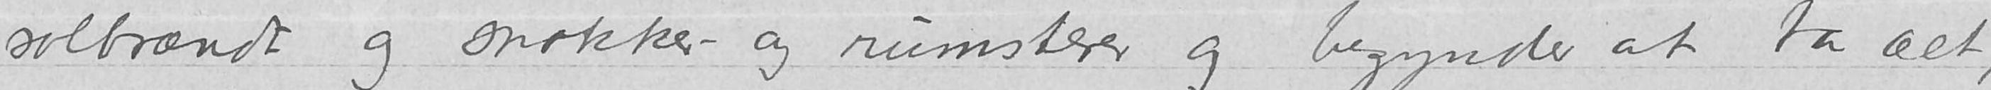solbrændt og snakker og rumsterer og begynder at ta alt,
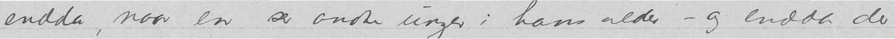endda, naar en ser andre unger i hans alder – og endda der
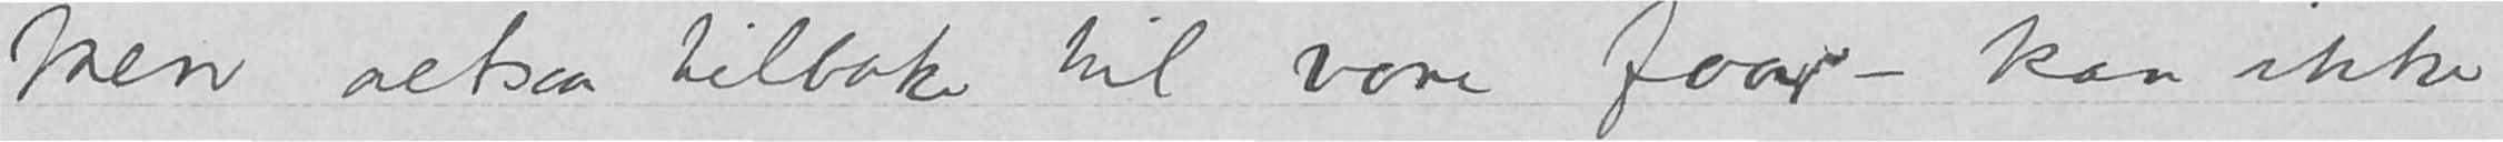Men altsaa tilbake til vore faar – kan ikke
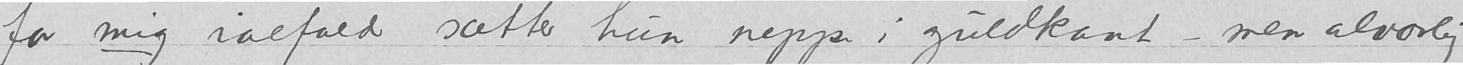for mig ialfald sætter hun neppe i guldkant – men alvorlig
